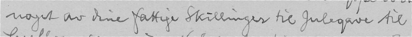noget av dine fattige Skillinger til Julegave til
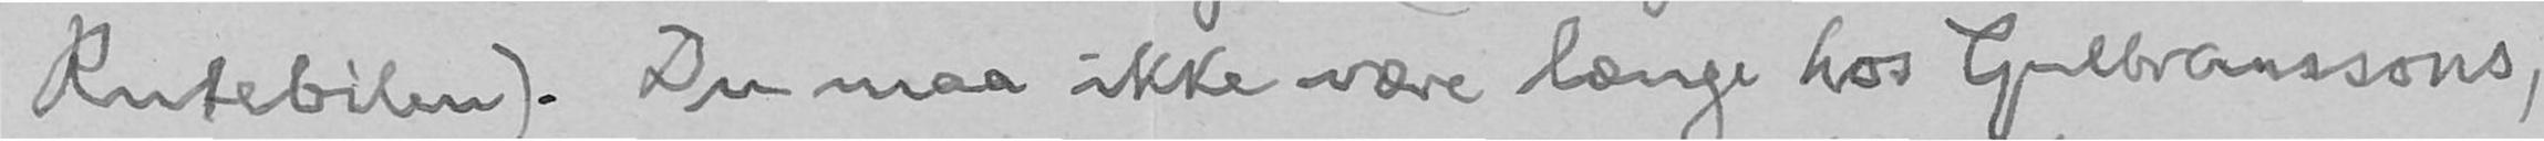Rutebilen). Du maa ikke være længe hos Gulbranssons,
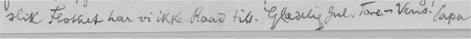slik Flothet har vi ikke Raad til. Glædelig Jul, Tore-Vens! Papa
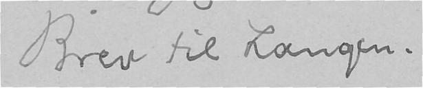Brev til Langen.
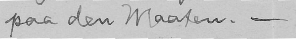paa den Maaten. -
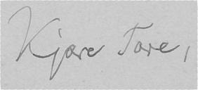Kjære Tore,
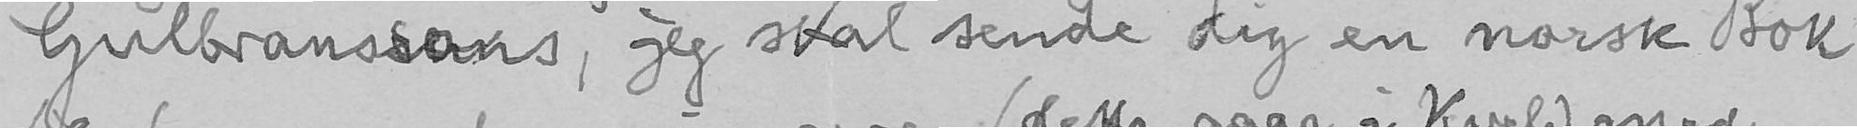Gulbranssons, jeg skal sende dig en norsk Bok
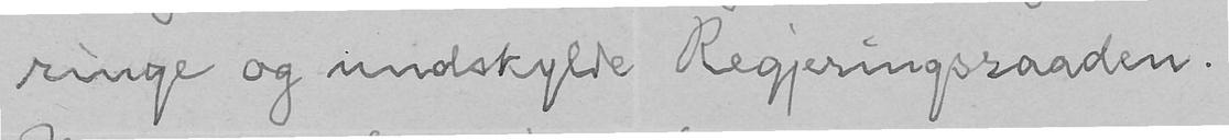ringe og undskylde Regjeringsraaden.
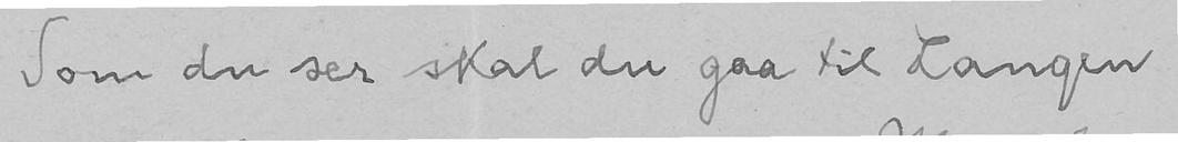Som du ser skal du gaa til Langen
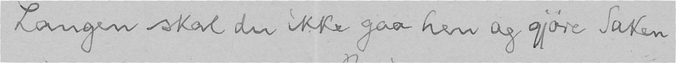Langen skal du ikke gaa hen og gjøre Saken
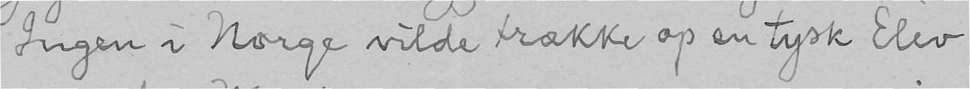Ingen i Norge vilde trække op en tysk Elev
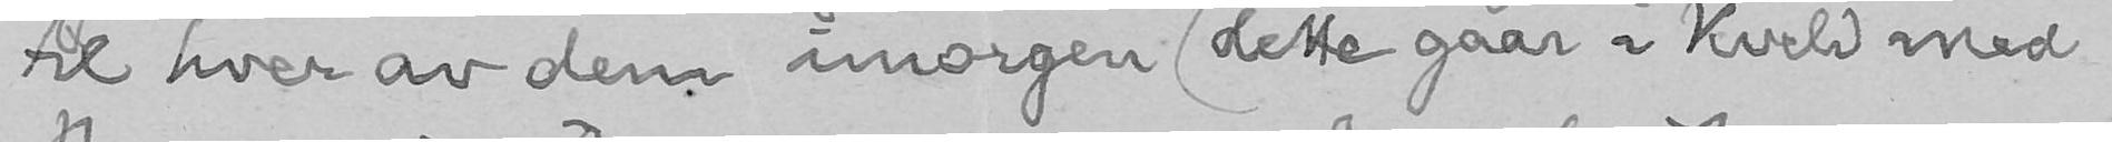til hver av dem imorgen (dette gaar i Kveld med
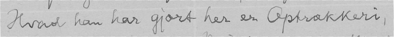Hvad han har gjort her er Optrækkeri,
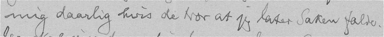mig daarlig hvis de tror at jeg later Saken falde.
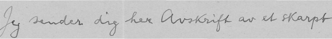Jeg sender dig her Avskrift av et skarpt
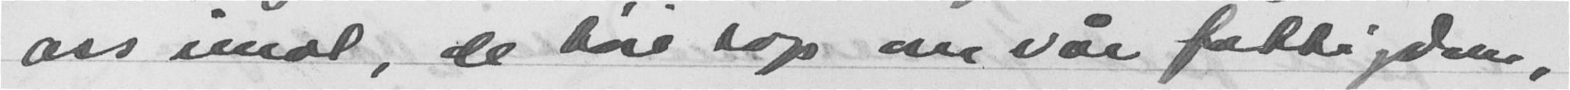oss imot, de høie rop om vår fattigdom,
